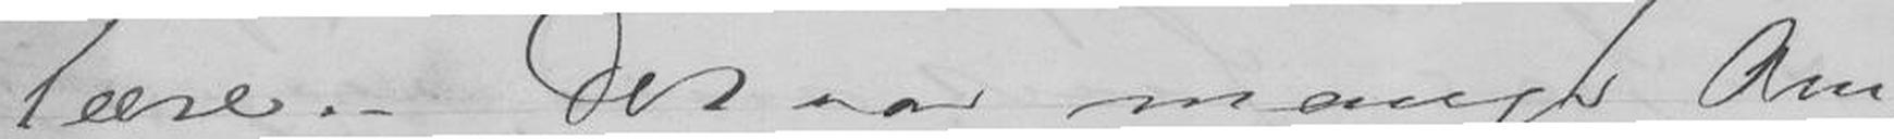lære. - Det var mange Om-
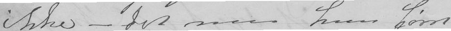ikke - det maa hun først
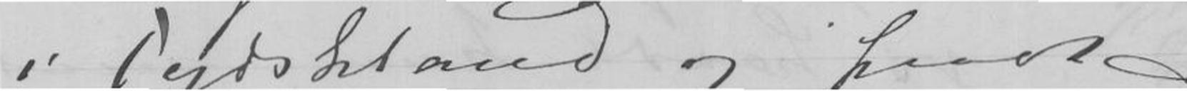i Tydskland og faaet
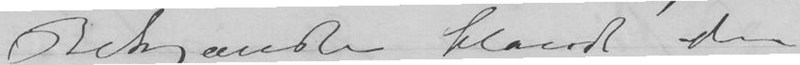Bekjændte blandt den
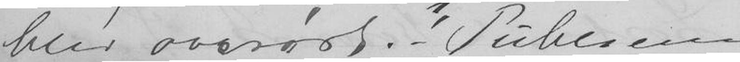blev overøst 1, Publicum
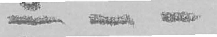- - -
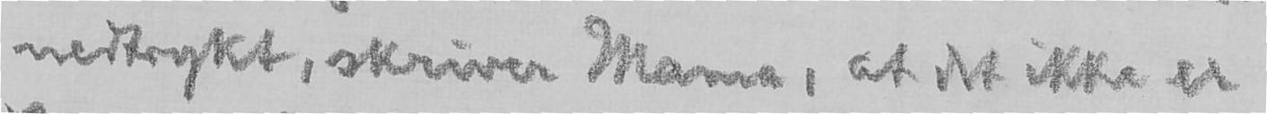nedtrykt, skriver Mama, at det ikke er
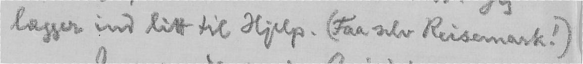lægger ind litt til Hjelp. (Faa selv Reisemark!)
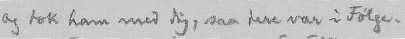og tok ham med dig, saa dere var i Følge.
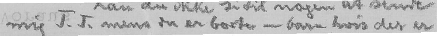mig T. T. mens du er borte - bare hvis der er
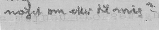noget om eller til mig?
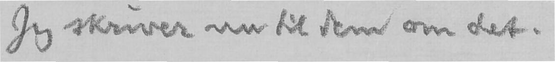Jeg skriver nu til dem om det.
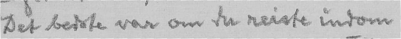Det bedste var om du reiste indom
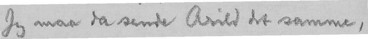Jeg maa da sende Arild det samme,
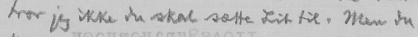tror jeg ikke du skal sætte Lit til. Men du
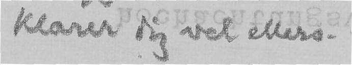klarer dig vel ellers.
